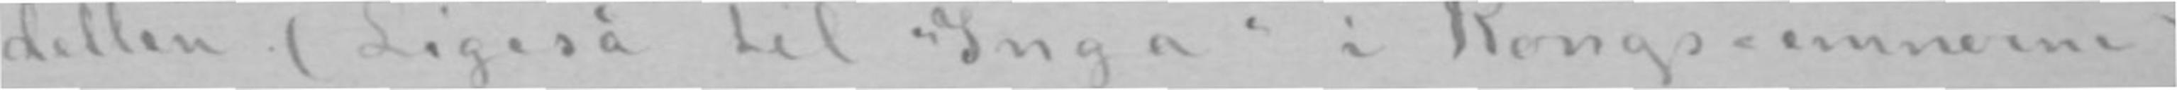dellen. (Ligeså til «Inga» i Kongs-emnerne)
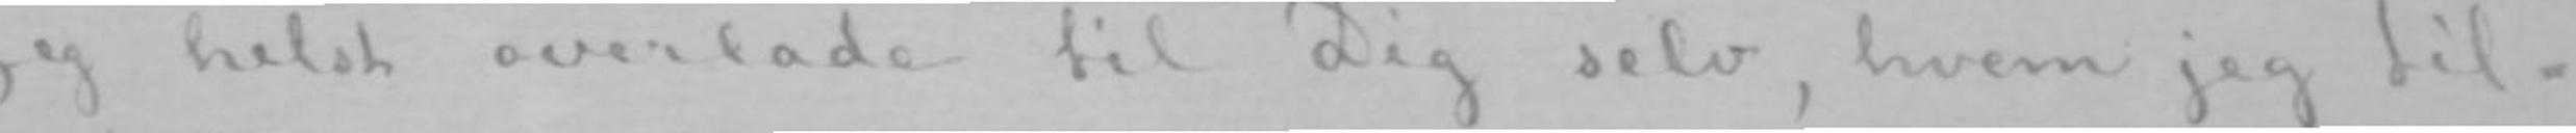jeg helst overlade til Dig selv, hvem jeg til-
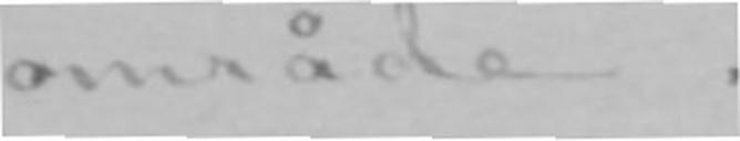område.
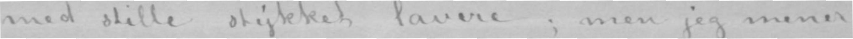med stille stykket lavere; men jeg mener
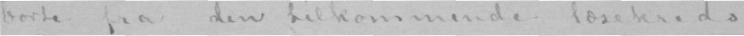borte fra den tilkommende læsekreds
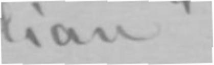lian».
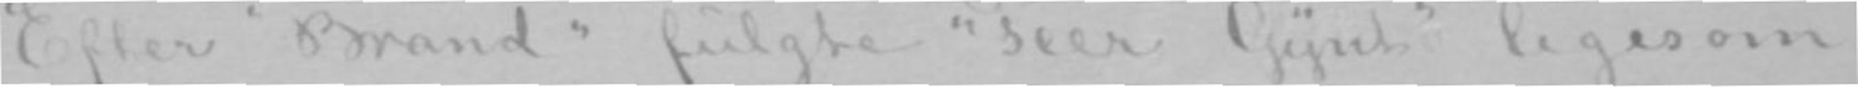Efter «Brand» fulgte «Peer Gynt» ligesom
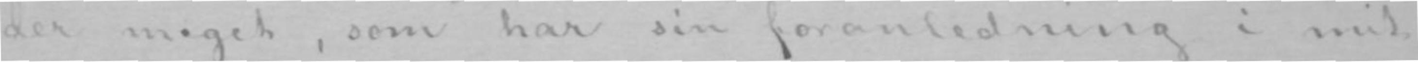der meget, som har sin foranledning i mit
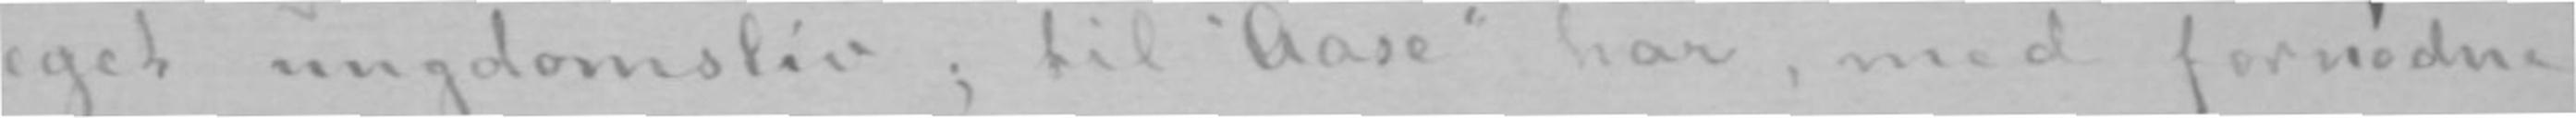eget ungdomsliv; til «Aase» har, med fornødne
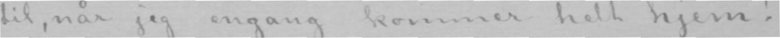til, når jeg engang kommer helt hjem!
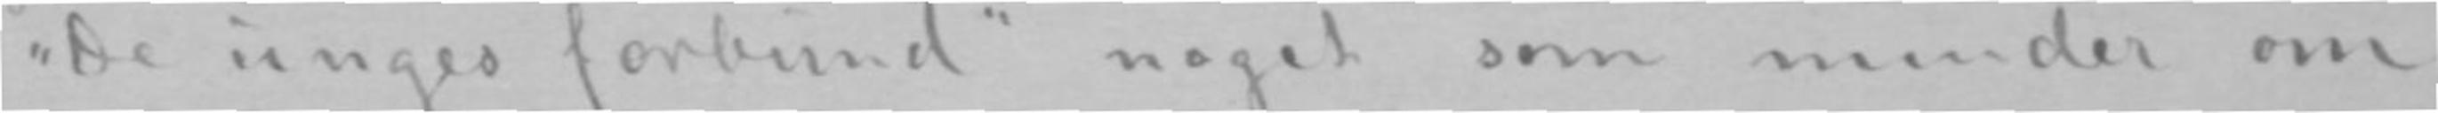i «De unges forbund» noget som minder om
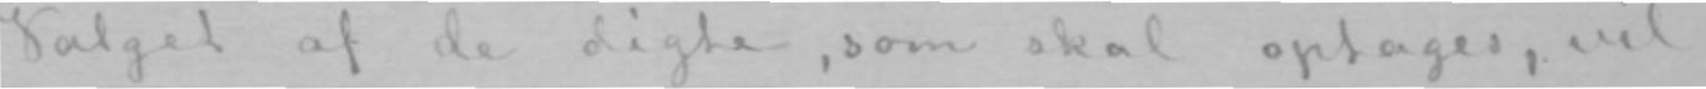Valget af de digte, som skal optages, vil
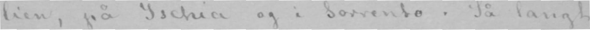lien, på Ischia og i Sorrento. Så langt
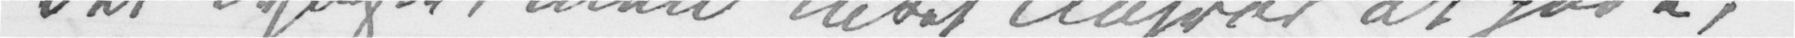det dybeste, men intet Ansvar at have,
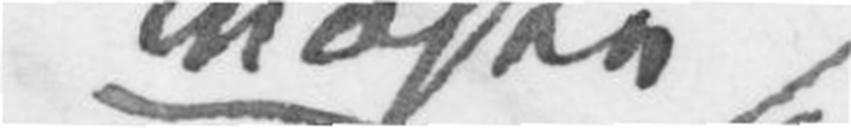måske
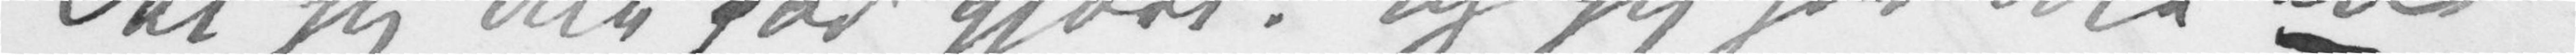det jeg ikke har gjort, og jeg har ikke en
